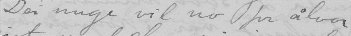Dei unge vil no for ålvor
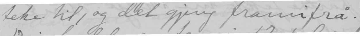teke til, og det gjeng framifrå.
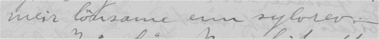meir lönsame enn sylvrev.
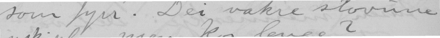som fyrr. Dei vakre stovune
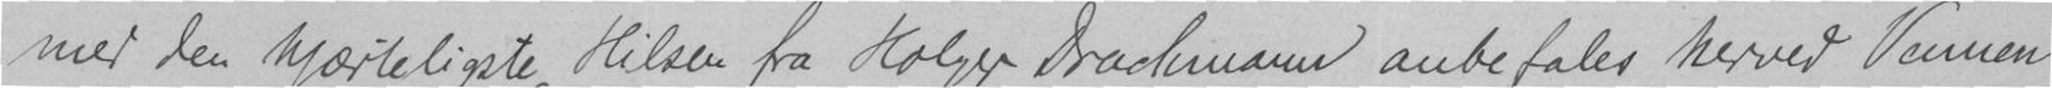med den hjærteligste Hilsen fra Holger Drachmann anbefales herved Vennen
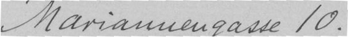Mariennengasse 10.
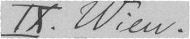IX Wien
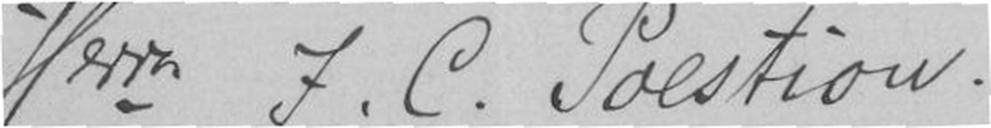Herrn I.C. Poestiow
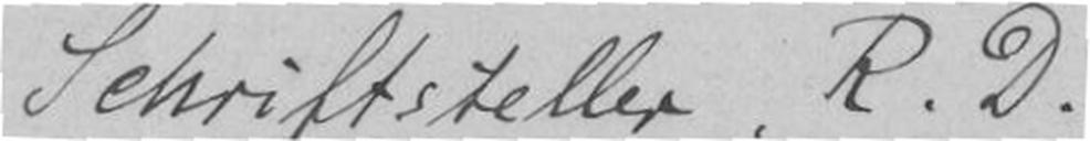Schriftsteller R.D.
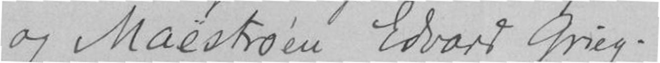og Maestro'en Edvard Grieg.
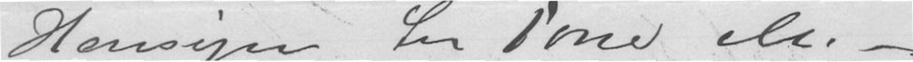Hensyn til Tone els. -
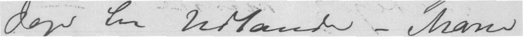Dage til Udlandet - Marie
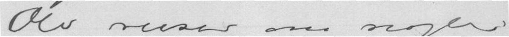Ole reiser om nogle
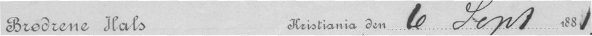Brødrene Hals Kristiania den 5 Sept 1881.
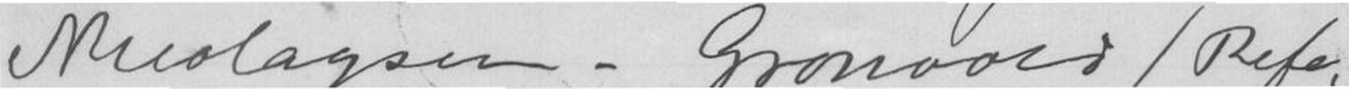Nicolaysen - Grøndvolg /Refe
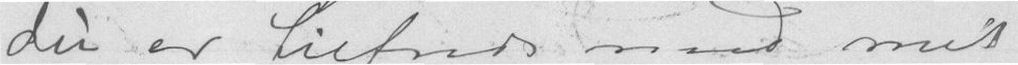Du er tilfreds med mit
